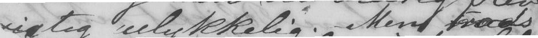igtig ulykkelig. Men trods
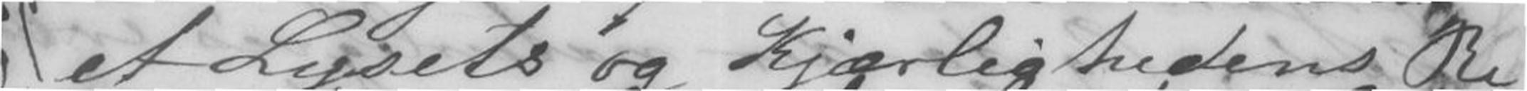et Lysets og Kjærlighedens Ri-
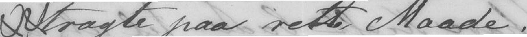tragte paa rette Maade. -
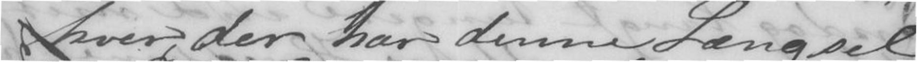hver der har denne Længsel
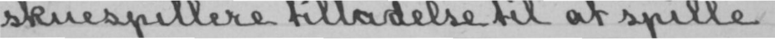skuespillere tilladelse til at spille
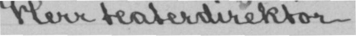Herr teaterdirektør
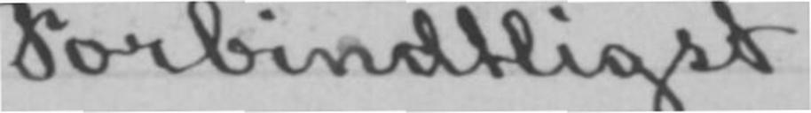Forbindtligst
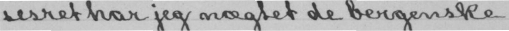sesret har jeg nægtet de bergenske
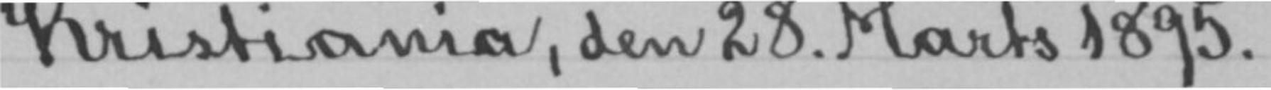Kristiania, den 28. Marts 1895.
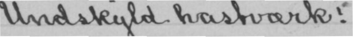Undskyld hastværk!
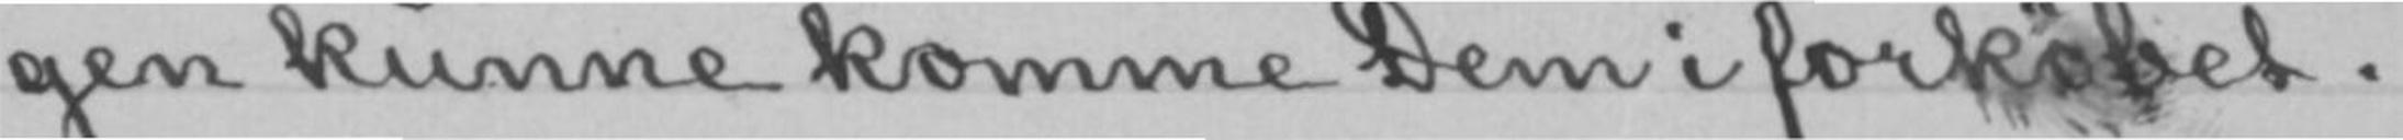gen kunne komme Dem i forkøbet.
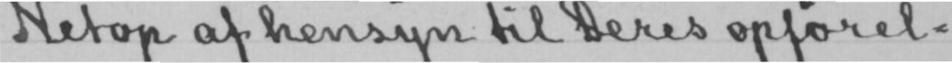Netop af hensyn til Deres opførel-
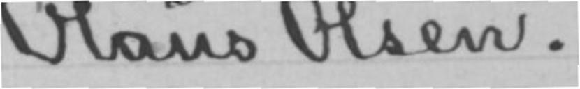Olaus Olsen.
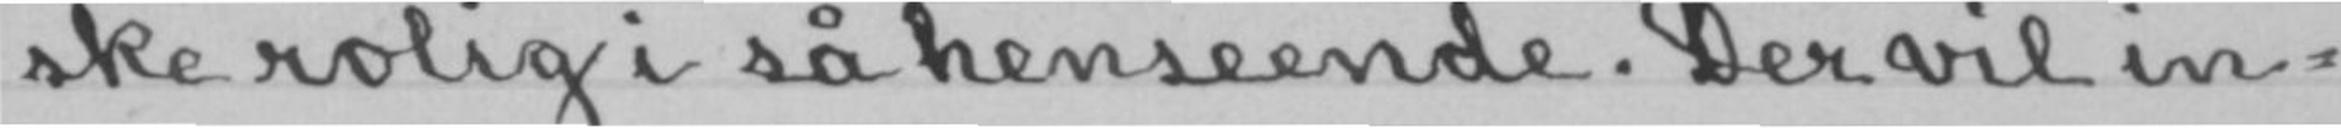ske rolig i så henseende. Der vil in-
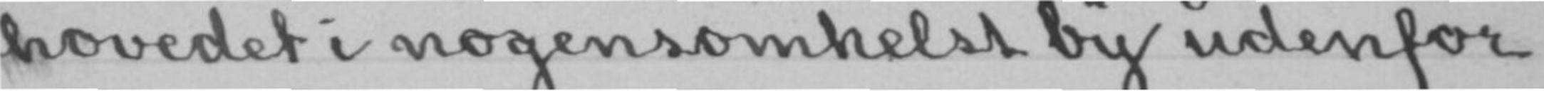hovedet i nogensomhelst by udenfor
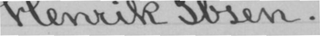Henrik Ibsen.
